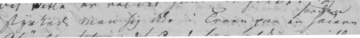styrkede man sig ikke i Troen paa en saadan høiere
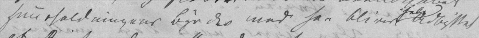Huusholdningens Byrder med saa bliver hele Udbyttet
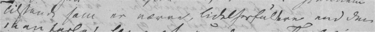Tilstande som er værre, lidelsesfuldere end dem
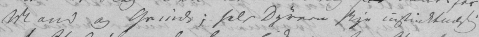Mand og Qvinde; selv Dyrene skye instinktmæssig
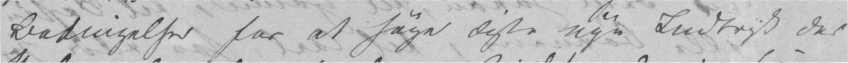Betingelser for at søge disse nye Indtryk der
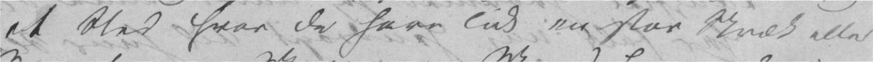et Sted hvor de have lidt en stor Skræk eller
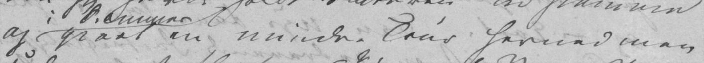og Sommer gjort en mindre Tour herned men
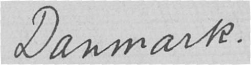Danmark.
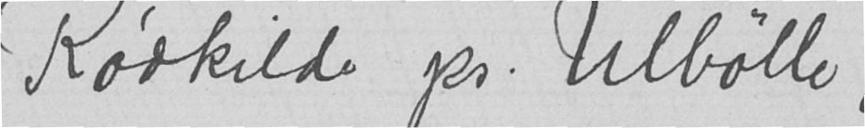Rødkilde pr. Ulbølle,
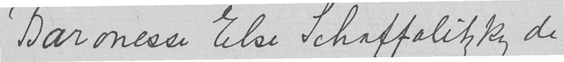Baronesse Else Schaffalitzky de
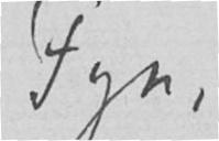Fyn,
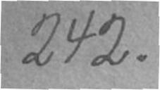242.
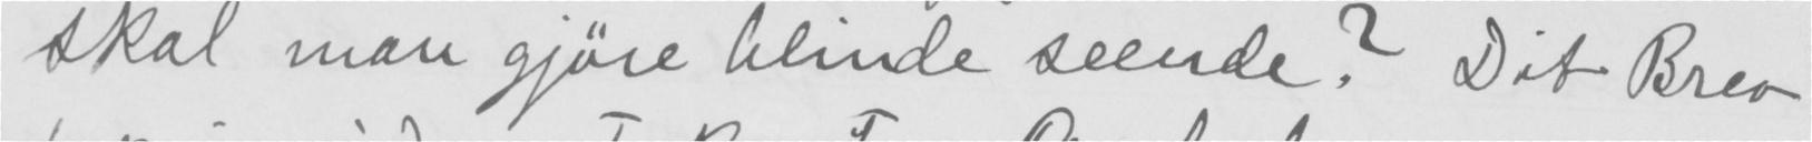skal man gjøre blinde seende? Dit Brev
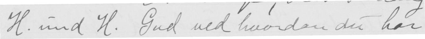H. und H. Gud ved hvordan du har
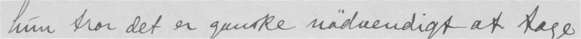hun tror det er ganske nødvendigt at tage
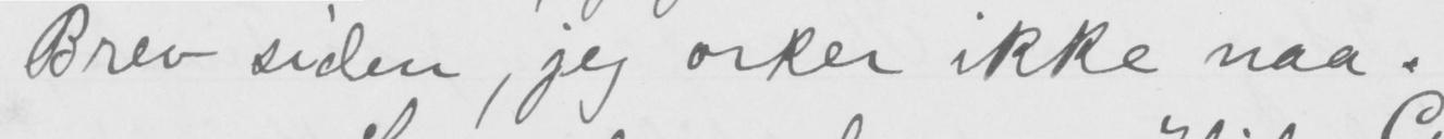Brev siden, jeg orker ikke naa.
